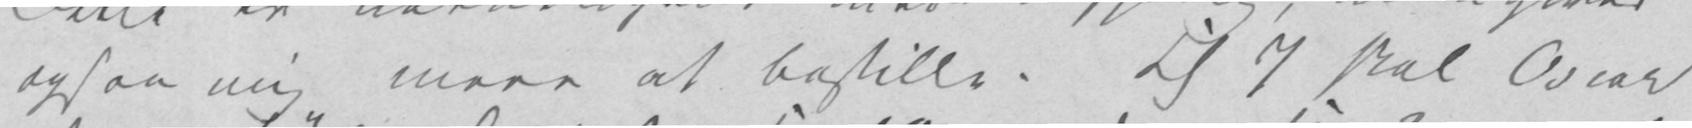ogsaa mig mere at bestille. Kl 7 skal Oscar
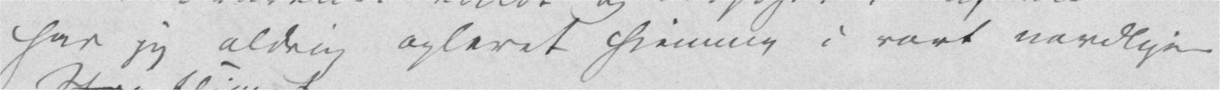har jeg aldrig oplevet hjemme i vort nordligere
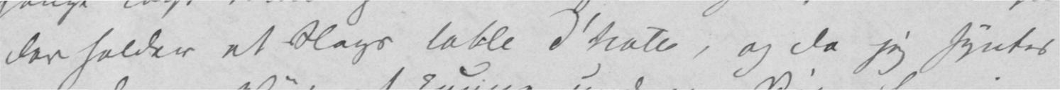der holder et Slags table d'hote, og da jeg syntes
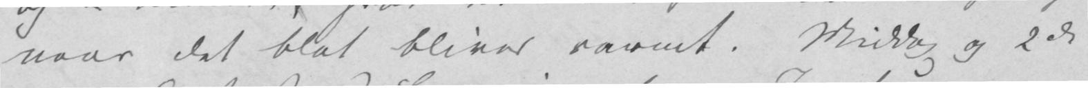naar det blot bliver varmt. Middag og tvende
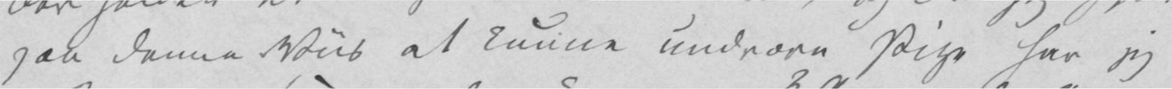paa denne Viis at kunne undvære Pige har jeg
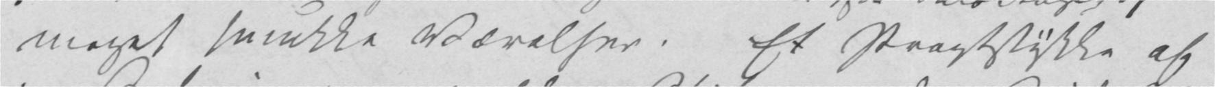meget smukke Værelser. Et Pragtstykke af
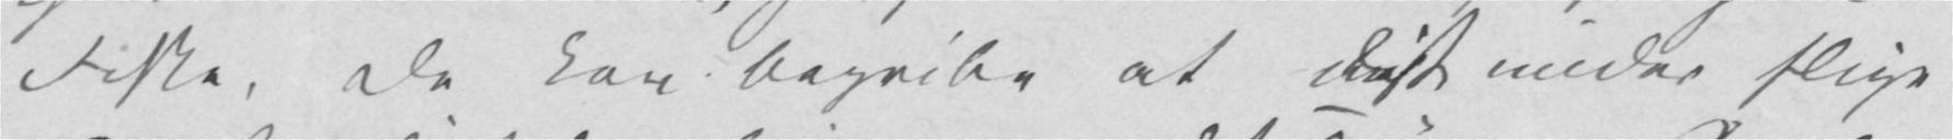Fiske, De kan begribe at disse under slige
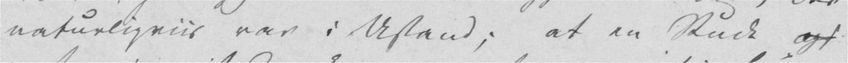naturligviis var i Ustand; at en Rude ogs
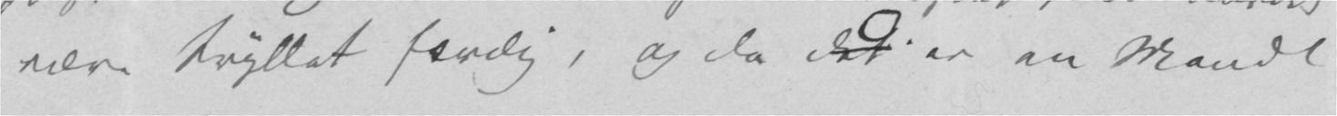være tryllet færdig, og da det O. er en Mand
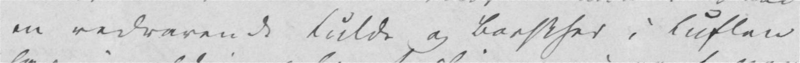en vedvarende Kulde og Barskhed i Luften
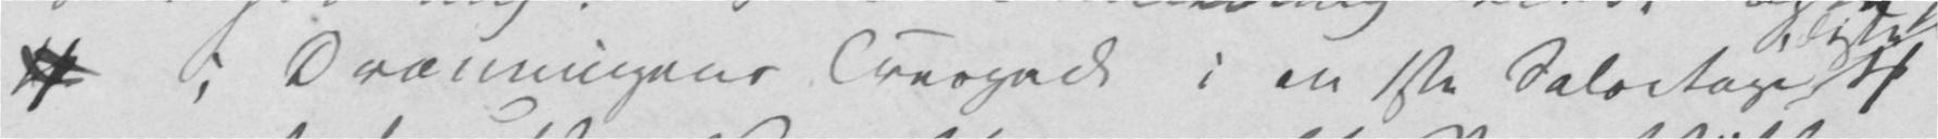4 i Dronningens Tvergade i en 1ste Salsetage disse 4
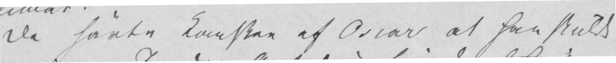De hørte kanskee af Oscar at han skulde
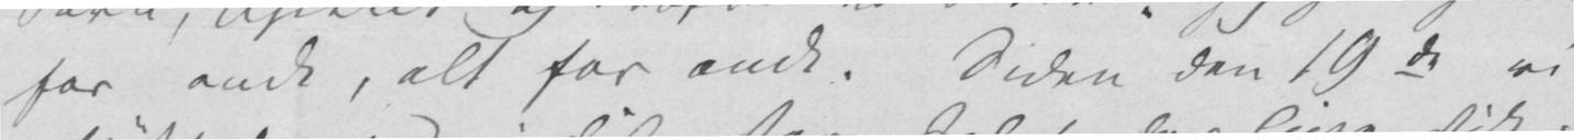for ondt, alt for ondt. Siden den 19de vi
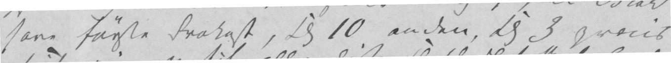have første Frokost, kl 10 anden, kl 3 præcis
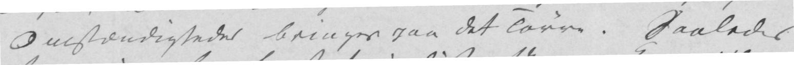Omstændigheder bringes paa det Tørre. Saaledes
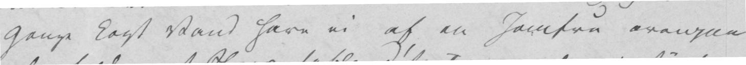Gange kogt Vand have vi af en Jomfru ovenpaa
